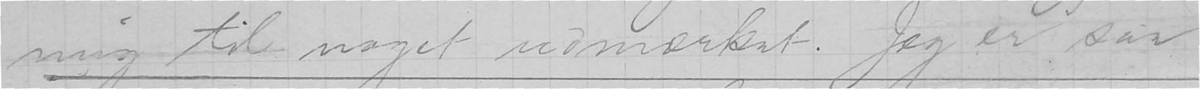mig til noget udmærket. Jeg er saa
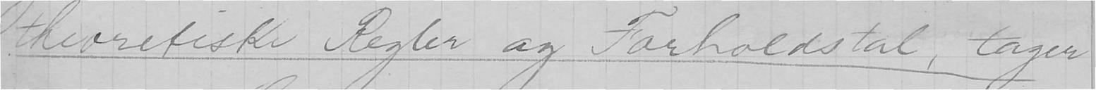theoretiske Regler og Forholdstal, tager
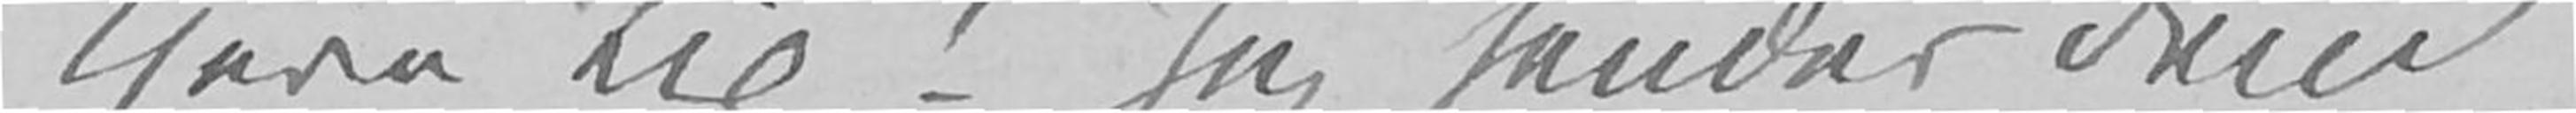Kjere Lie! Jeg sender Dem
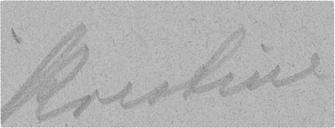Kristine
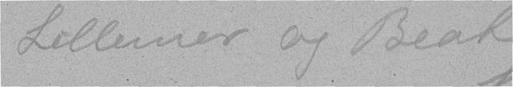Lillemor og Beate
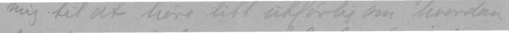mig til at høre litt utførlig om hvordan
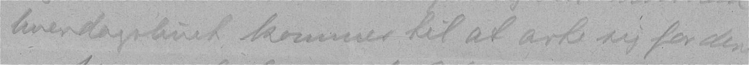hverdagslivet kommer til at arte sig for dere.
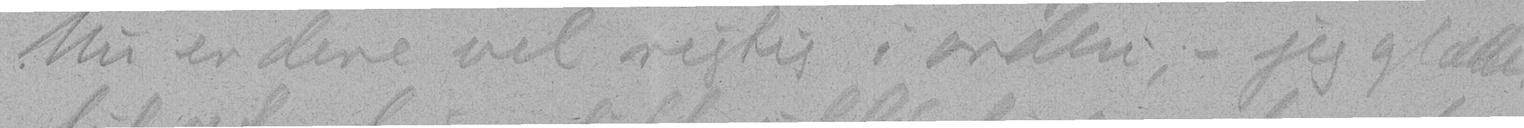Nu er dere vel rigtig i orden, - jeg glæder
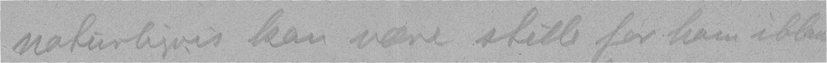naturligvis kan være stille for ham iblandt.
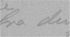fra din
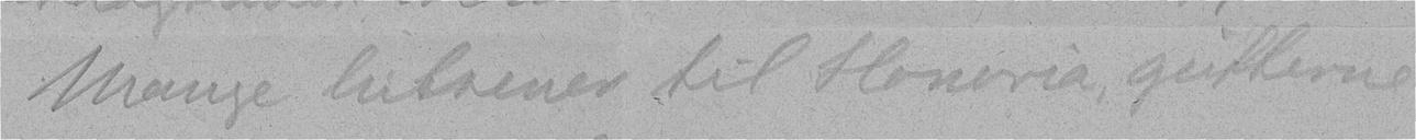Mange hilsener til Honoria, gutterne
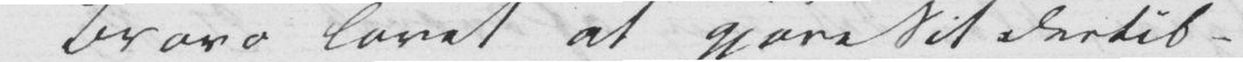Bravo lovet at gjøre Sit dertil.
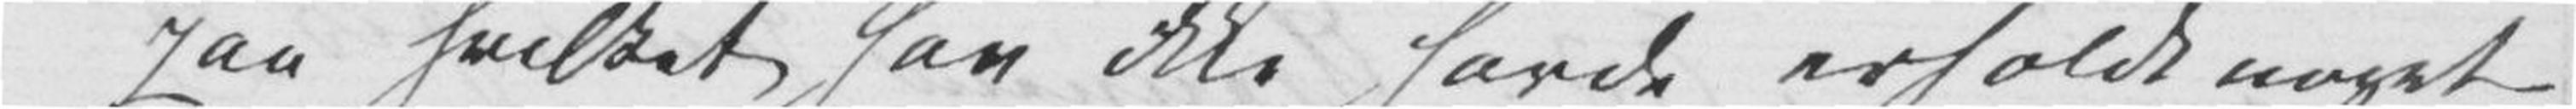paa hvilket han ikke havde erholdt noget
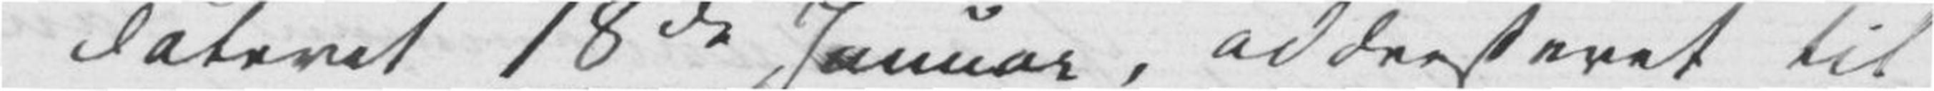dateret 18de Januar, addresseret til
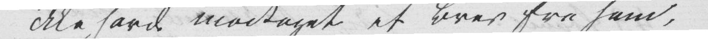ikke havde modtaget et Brev fra ham,
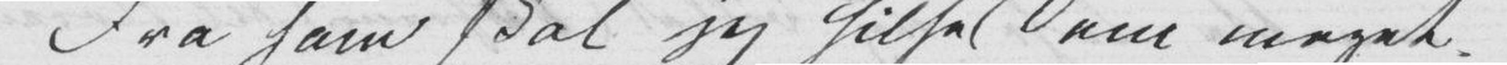Fra ham skal jeg hilse Dem meget.
